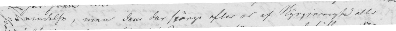vindelse, men dem der spørge efter os af Nysgjerrighed eller
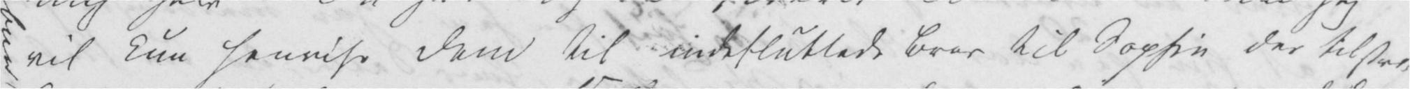vil kun henvise Dem til indesluttede Brev til Sophie der tilstræk
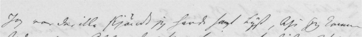Jeg var der ikke skjøndt jeg havde havt Lyst, thi jeg kommer
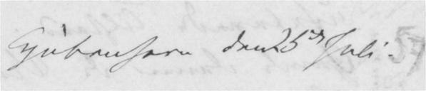Kjøbenhavn den 25de Juli.
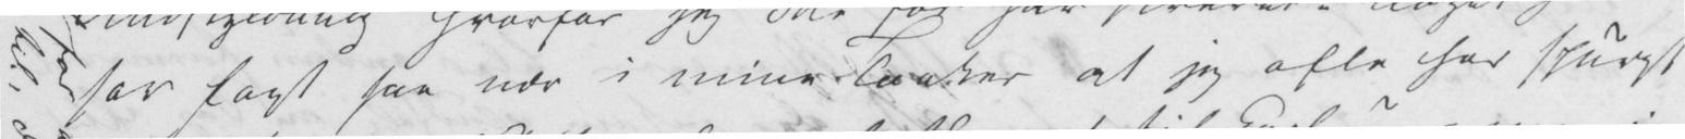har lagt saa nær i mine Tanker at jeg ofte har spurgt
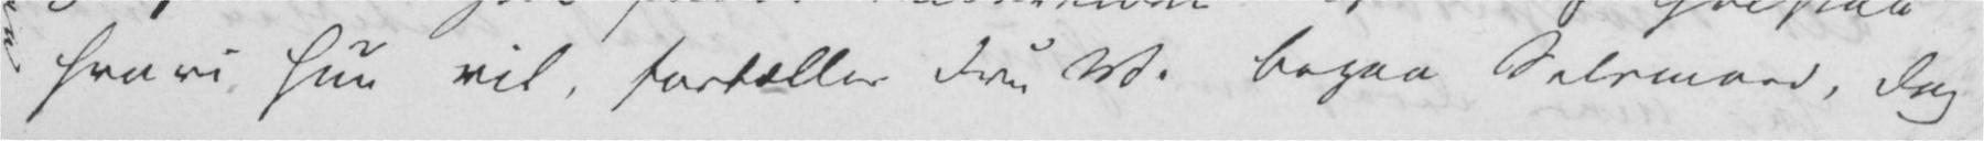hvori hun vil, fortæller Fru V. begaa Selvmord, dog
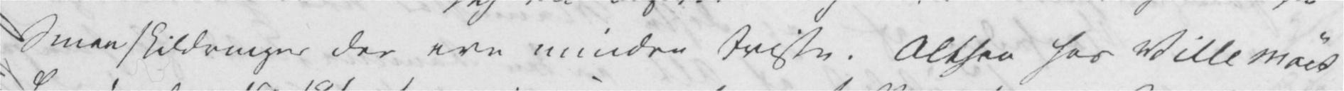Smaaskildringer der ere mindre triste. Altsaa hos Villemøes
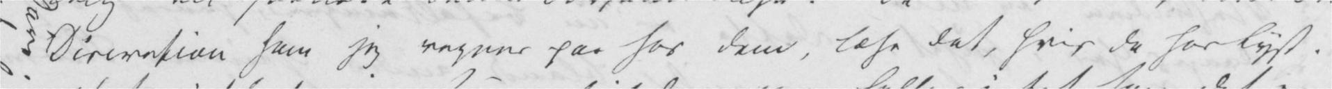Discretion som jeg regner paa hos Dem, læse det, hvis De har Lyst.
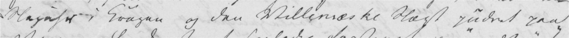Slaguhr i Krogen og den Villemœske Slægt pudret paa
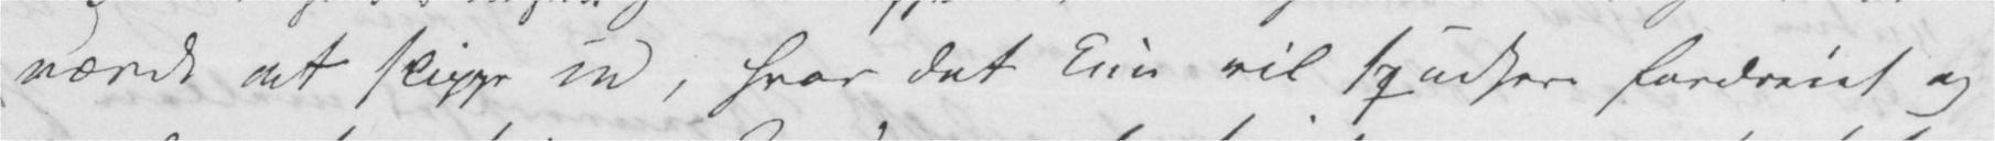værdt at slippe ud, hvor det kun vil spadsere fordreiet og
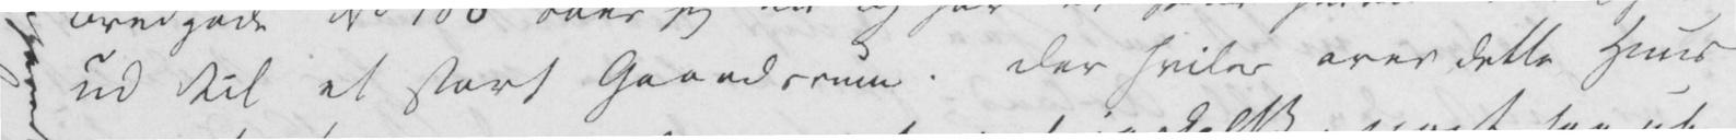ud til et stort Gaardsrum. Der hviler over dette Huus
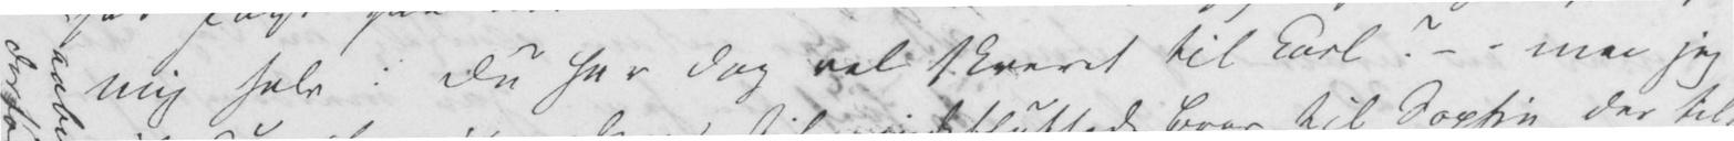mig selv: Du har dog vel skrevet til Carl? – – men jeg
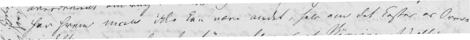for hvem man ikke kan være andet, selv om det koster os Over-
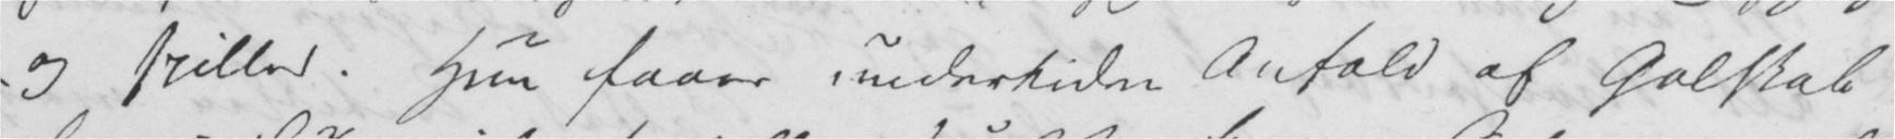og spiller. Hun faaer undertiden Anfald af Galskab
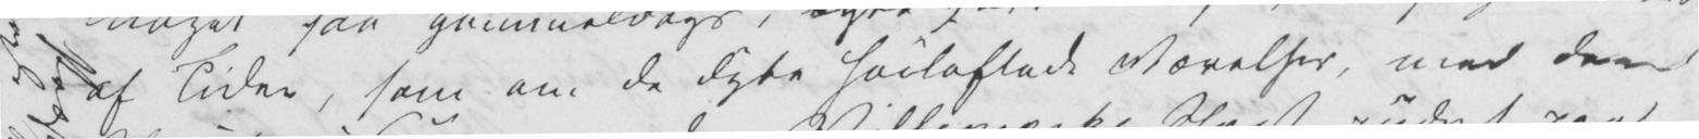af Tiden, som om de dybe høiloftede Værelser, med deres
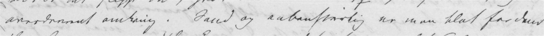overdrevent omkring. Sand og aabenhjertig er man blot for dem
